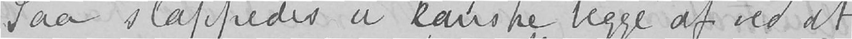Saa slappedes vi kanske begge af ved at
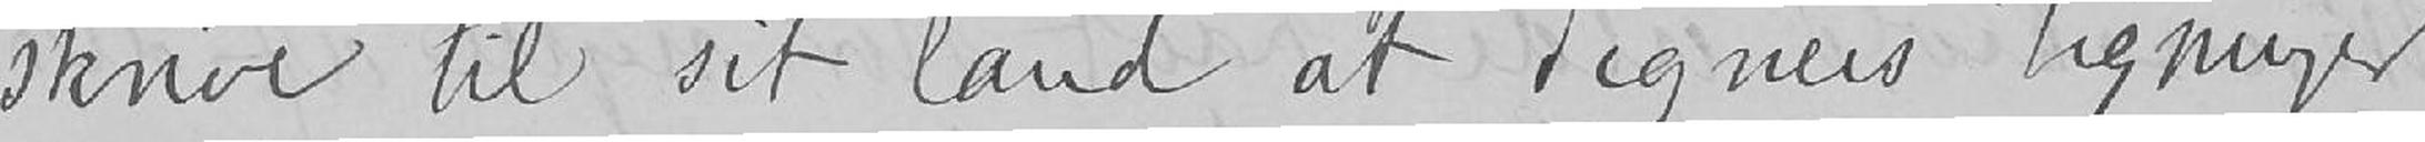skrive til sit land at Tegners tegninger
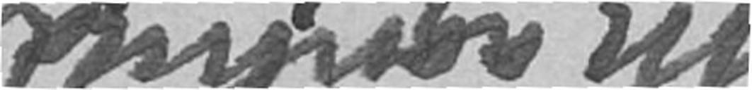Magnhild
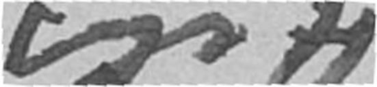Hils
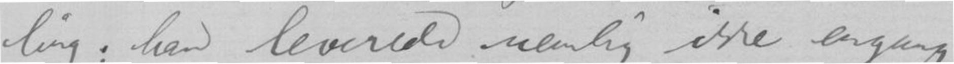ling; han leverde nemlig ikke engang
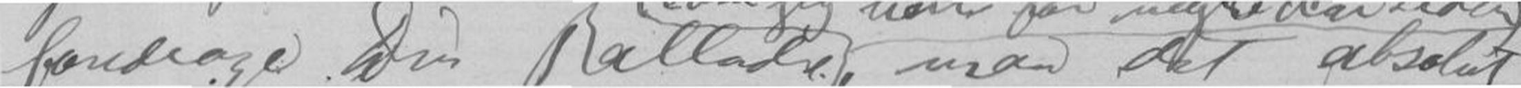foredrage Din Ballade, maa det absolut
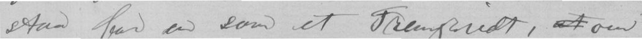staa for en som et Fremskridt, at om
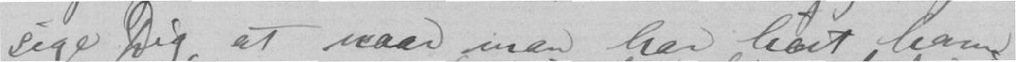sige Dig, at naar man har hørt ham
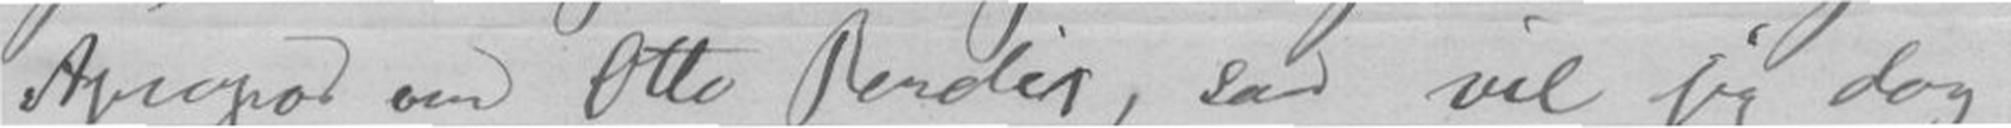Apropos om Otto Bendix, saa vil jeg dog
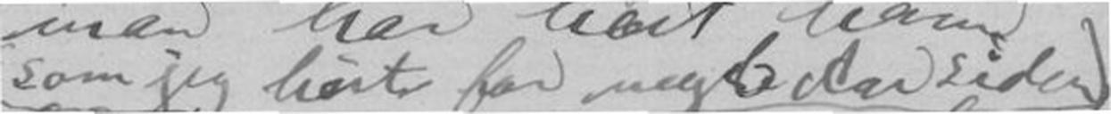som jeg hørte for nogle Aar siden
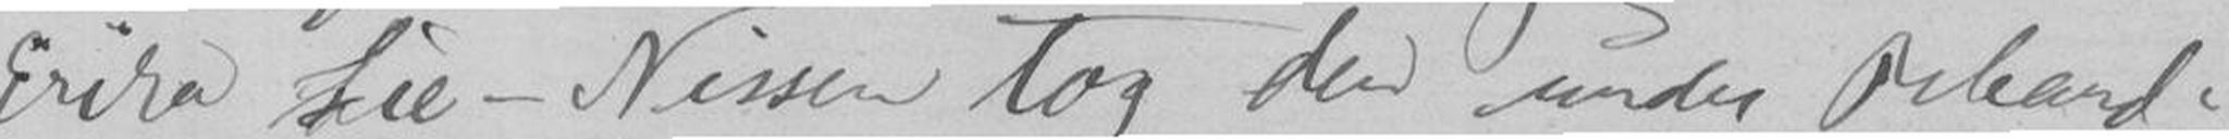Erika Lie-Nissen tog den under Behand-
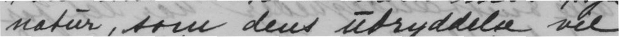natur, som dens udryddelse vil
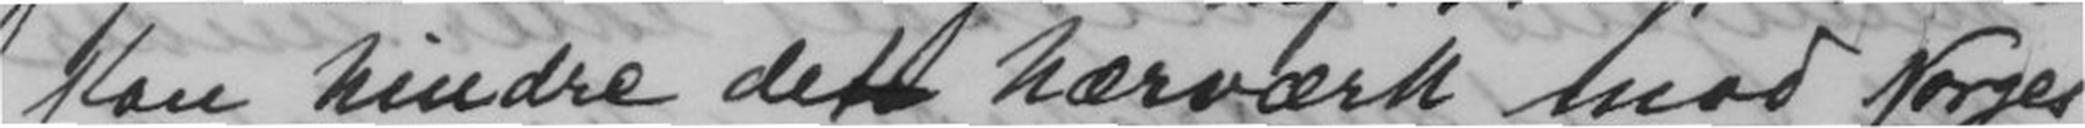kan hindre det hærværk mod Norges
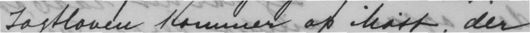Jagtloven kommer op ihøst, der
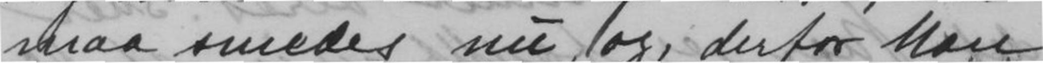maa smedes nu, og, derfor kan
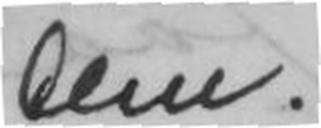Dem.
